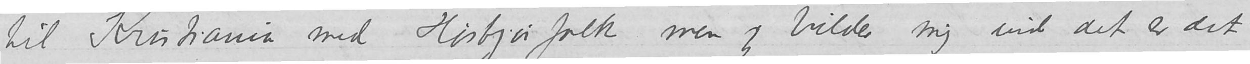til Kristiania med Høsbjørfolk men jeg bilder mig ind det er det
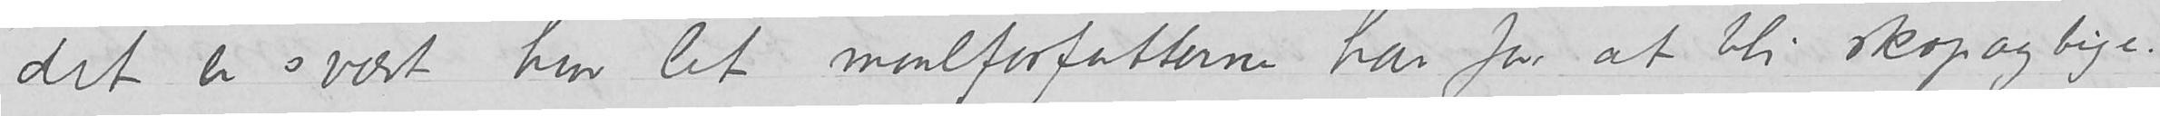det er svært hvor let maalforfatterne har for at bli skapaktige.
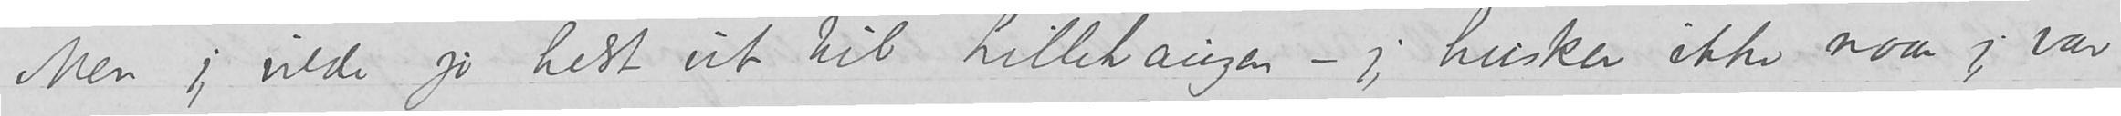Men jeg vilde jo helst ut til Lillehaugen – jeg husker ikke naar jeg var
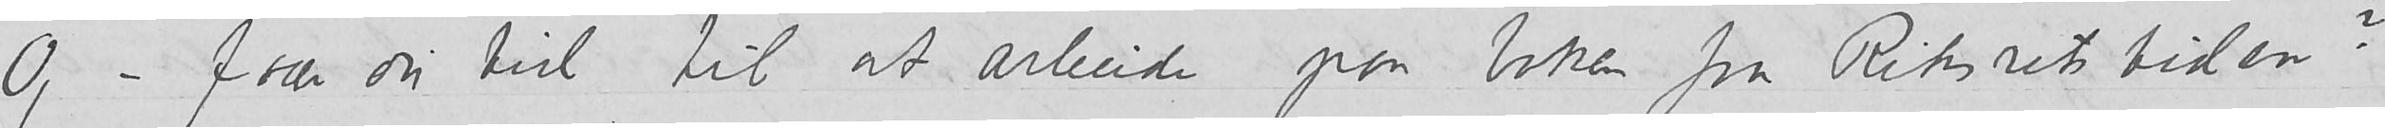Og – faar du tid til at arbeide paa boken fra Riksretstiden?
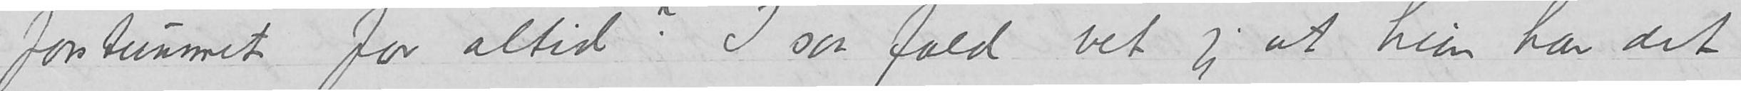forstummet for alltid? I saa fald vet jeg at hun har det
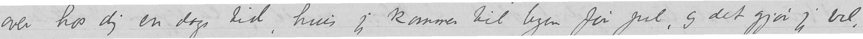over hos dig en dags tid, hvis jeg kommer til byen før jul, og det gjør jeg vel,
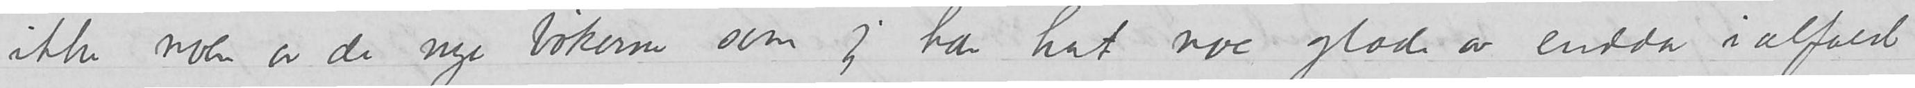ikke noen av de nye bøkerne som jeg har hat noe glæde av endda ialfald
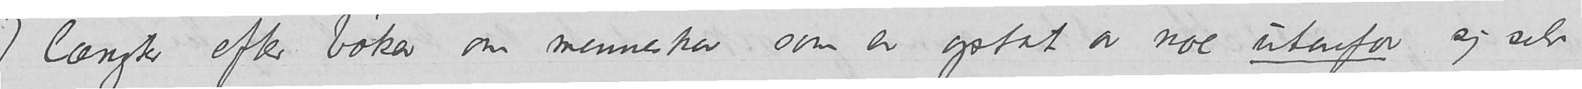Jeg længter efter bøker om mennesker som er optat av noe utenfor sig selv
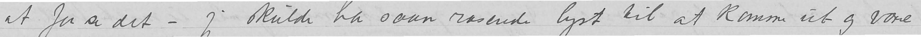at faa se det – jeg skulde ha saan rasende lyst til at komme ut og være
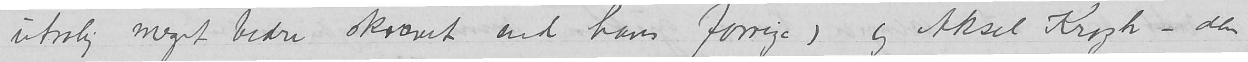utrolig meget bedre skrævet end hans forrige) og Aksel Krogh – den
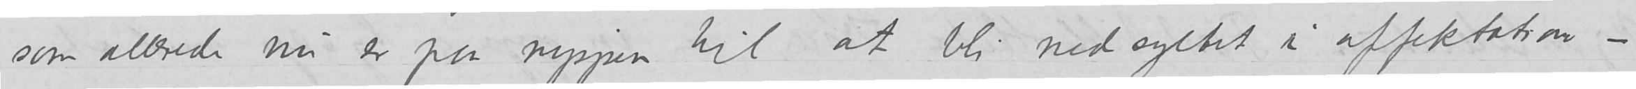som allerede nu er paa nippen til at bli nedsyltet i affektation –
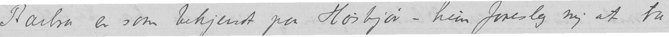Barbra er som bekjendt paa Høsbjør – hun foreslog mig at ta
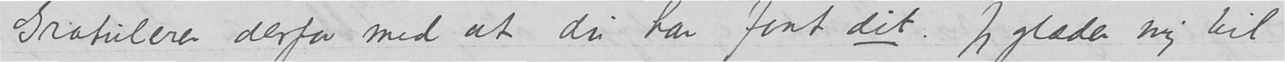Gratulerer derfor med at du har faat dit. Jeg glæder mig til
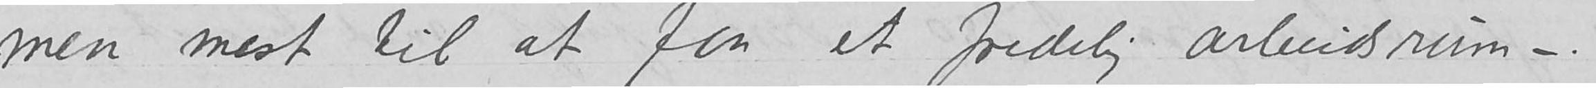men mest til at faa et fredelig arbeidsrum –.
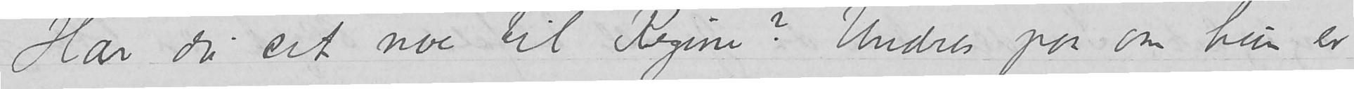Har du set noe til Regine? Undres paa om hun er
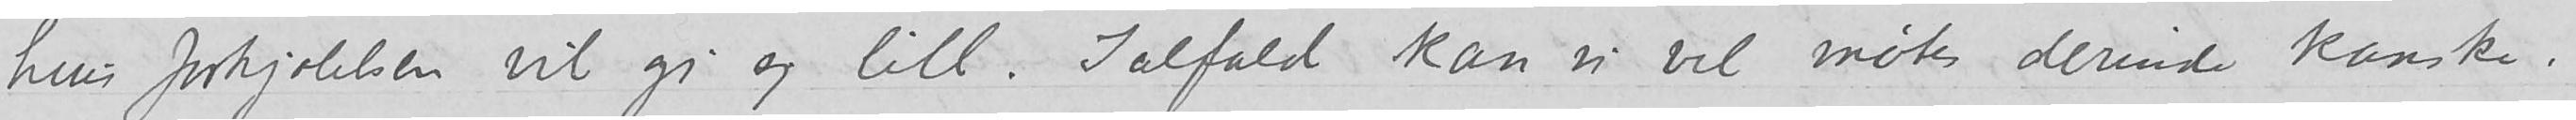hvis forkjølelsen vil gi sig litt. Ialfald kan vi vel møtes derinde kanske.
